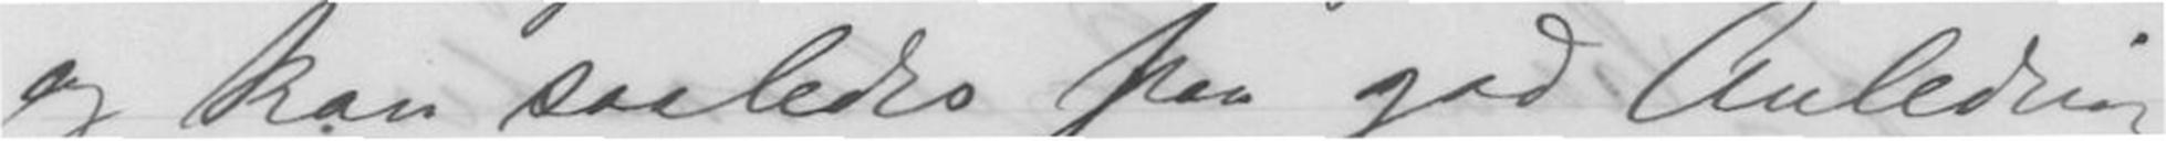og kan saaledes faa god Anledning
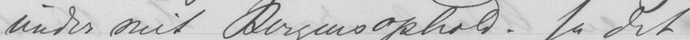under mit Bergensophold. Ja det
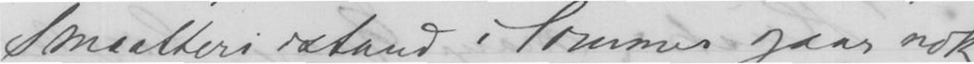Smaatteri istand i Sommer gaar nok
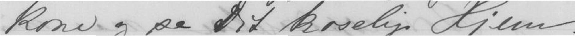Kone og se Dit koselige Hjem.
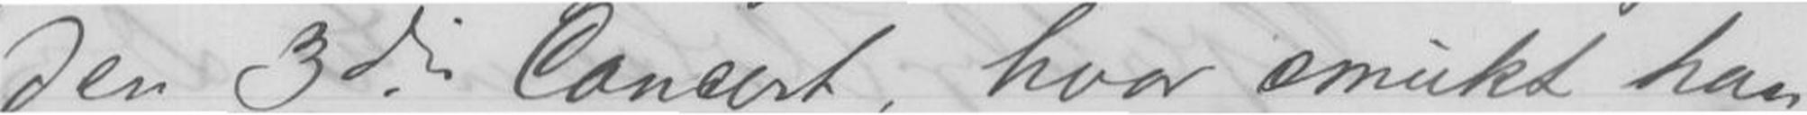den 3die Concert, hvor smukt han
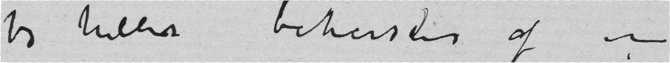jeg heller beherskes af en
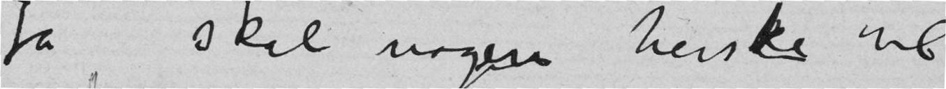Ja skal nogen herske vil
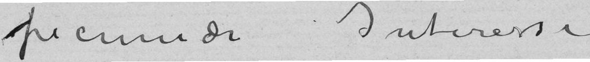fremmede Interesse
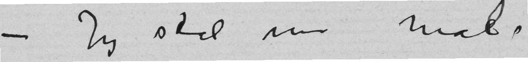– Jeg skal nu male
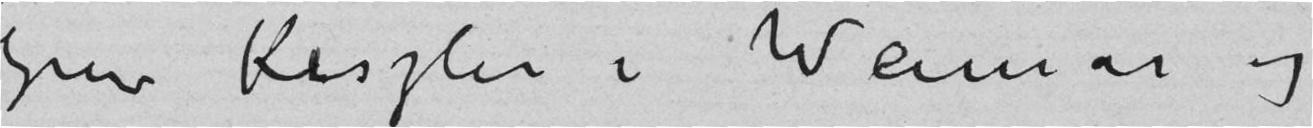Grev Kessler i Weimar og
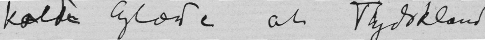kolde Glæde at Tydskland
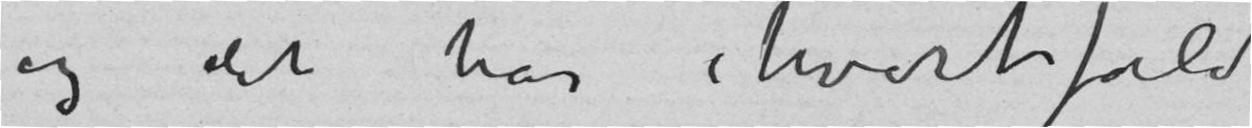og det har ihvertfald
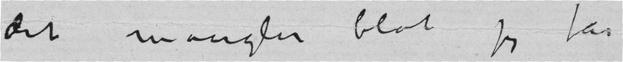det mangler blot jeg får
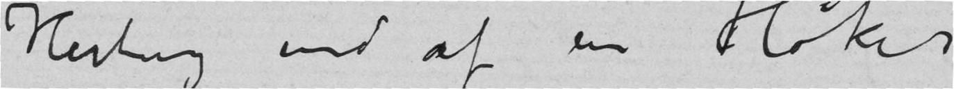Hertug end af en Høker
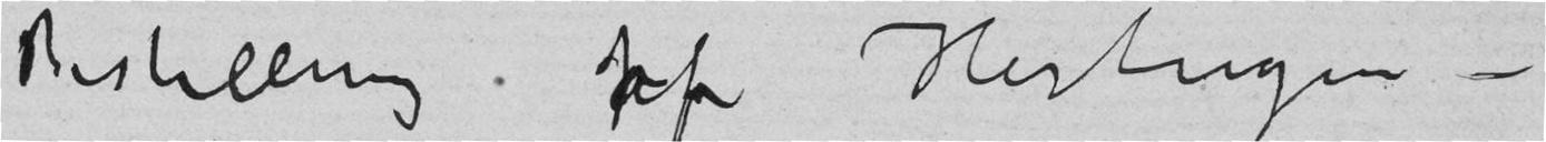Bestilling af Hertugen –
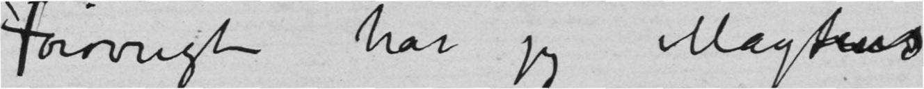Forøvrig har jeg Magtens
